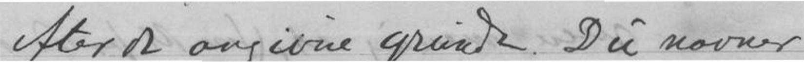efter de angivne Grunde. Du nævner
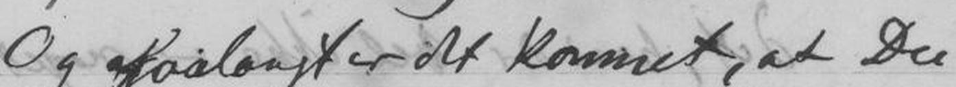Og saalangt er det kommet, at Du
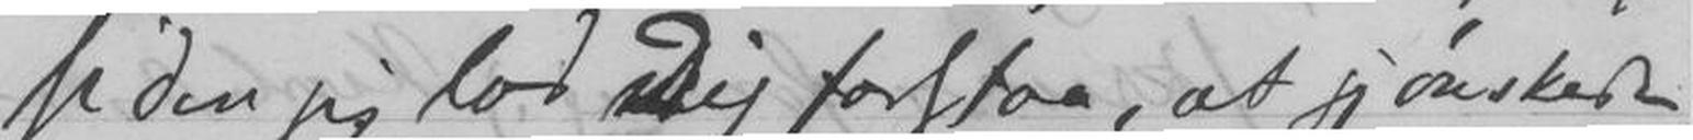siden jeg lod Dig forstaa, at jeg ønskede
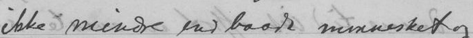ikke mindre end baade mennesket og
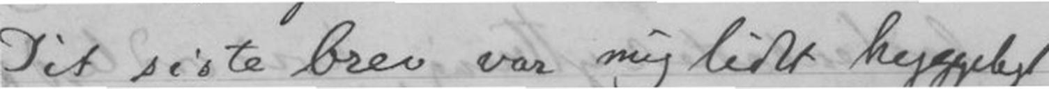Dit sidste brev var mig lidet hyggeligt
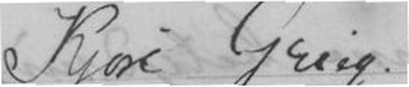Kjære Grieg.
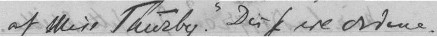af Miss Thusby." Disse ere Ordene.
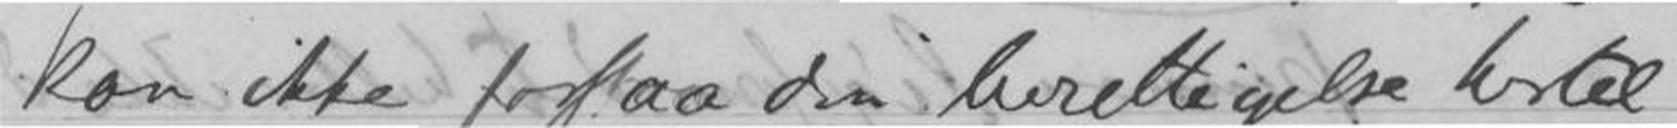kan ikke forstaa din berettigelse hertil
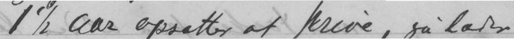1½ Aar opsætter at skrive, så lader
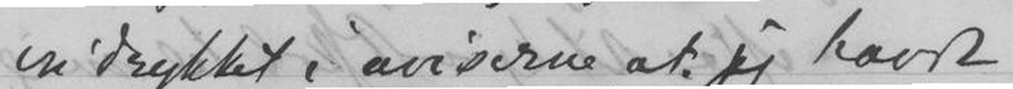indrykket i aviserne at jeg havde
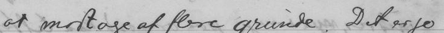at modtage af flere grunde. Det er jo
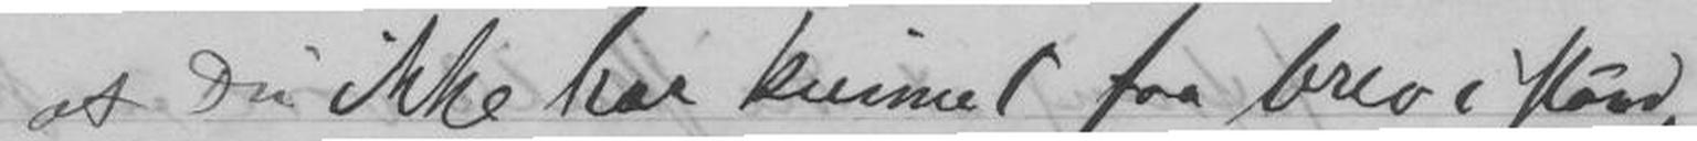at Du ikke har kunnet faa brev i stand,
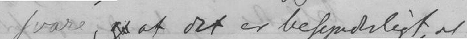svare, at det er besynderligt, at
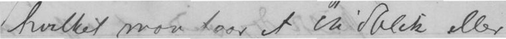hvilket man faar et indblik eller
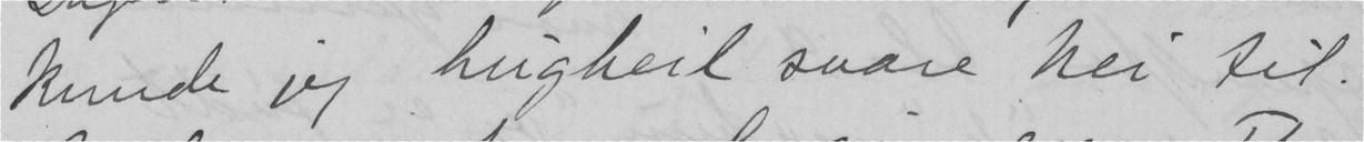kunde jeg hugheil svare nei til.
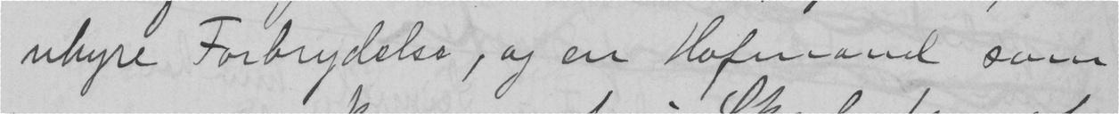uhyre Forbrydelse, og en Hofmand som
